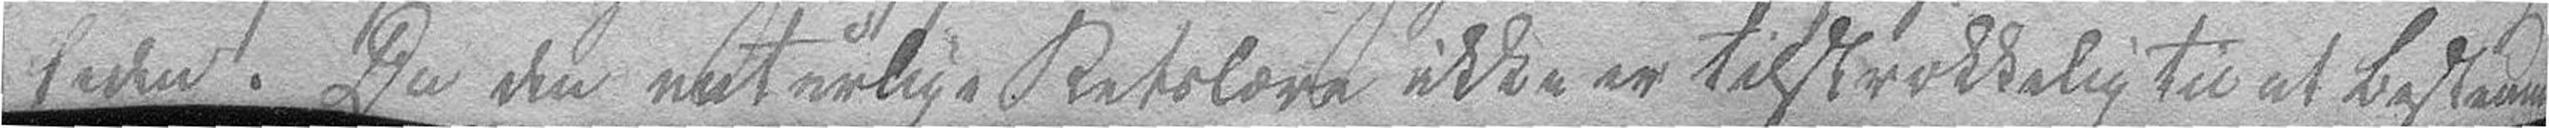heden. Da den naturlige Retslære ikke er tilstrækkelig til at bestemme
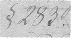§ 283.
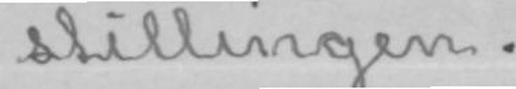stillingen.
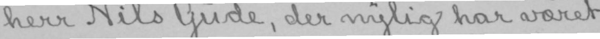herr Nils Gude, der nylig har været
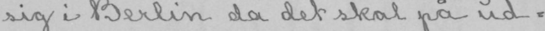sig i Berlin da det skal på ud-
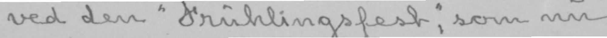ved den «Frühlingsfest», som nu
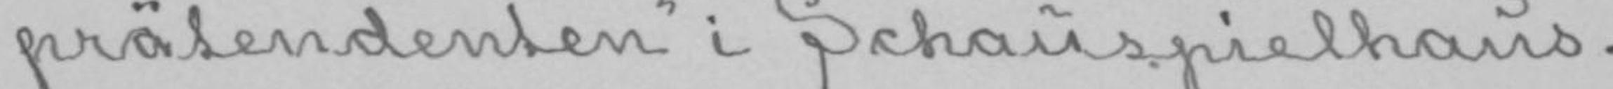prätendenten» i Schauspielhaus.
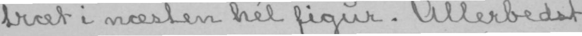træt i næsten hél figur. Allerbedst
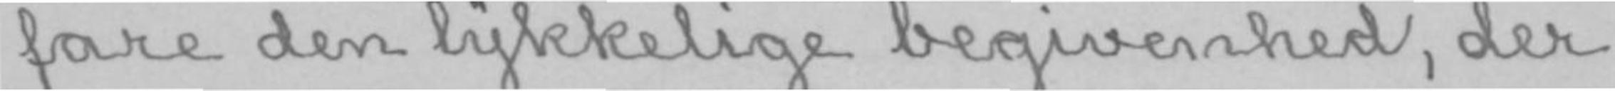fare den lykkelige begivenhed, der
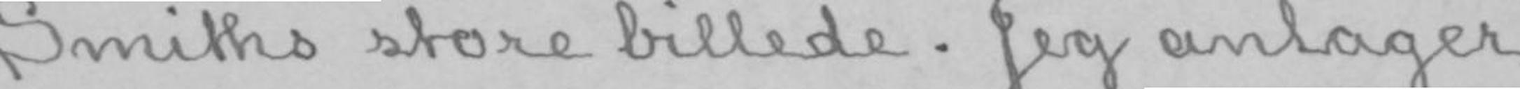Smiths store billede. Jeg antager
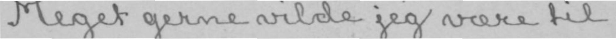Meget gerne vilde jeg være til-
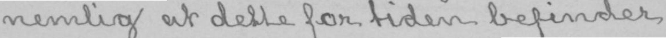nemlig at dette for tiden befinder
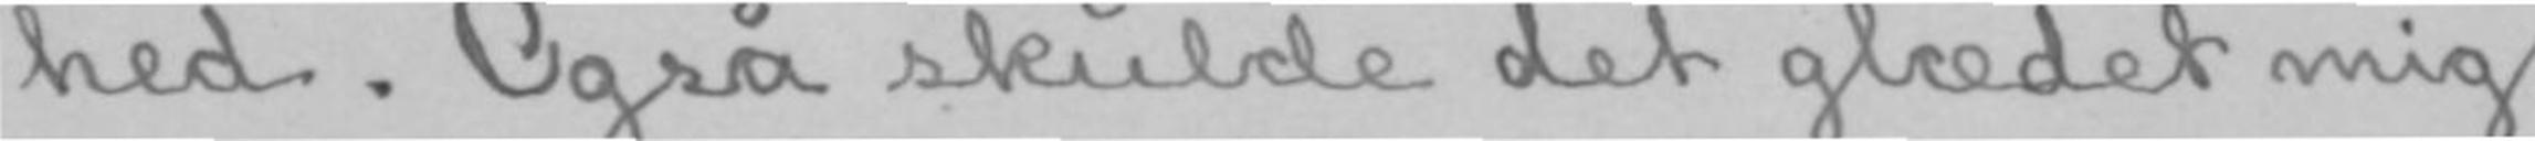hed. Også skulde det glædet mig
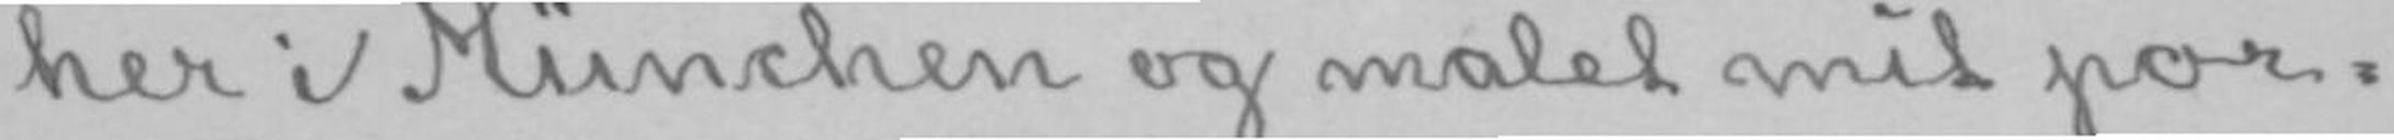her i München og malet mit por-
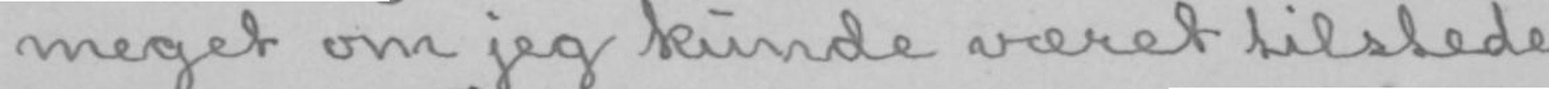meget om jeg kunde været tilstede
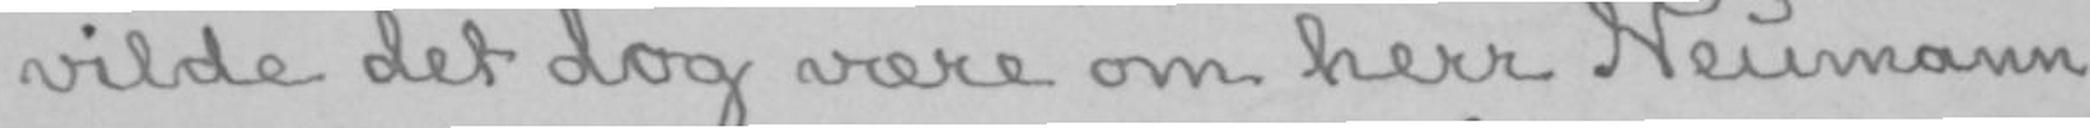vilde det dog være om herr Neumann
